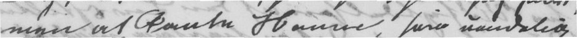men at Tante Hamme, saa uendelig
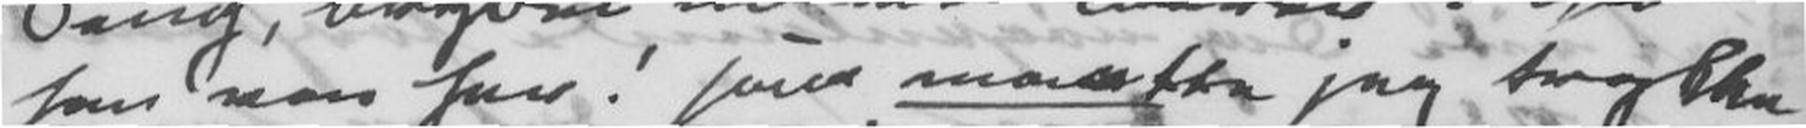han var for! saa maatte jeg trykke
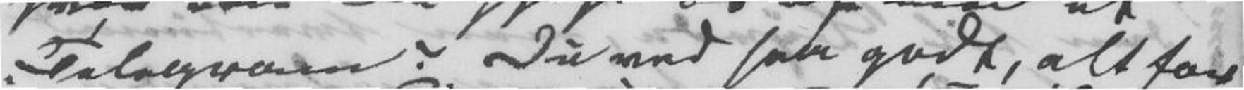Telegram? Du ved saa godt, alt for
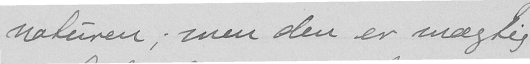naturen; men den er mægtig
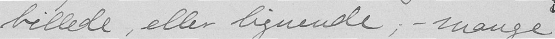billede, eller lignende, - mange
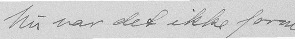Nu var det ikke formeget
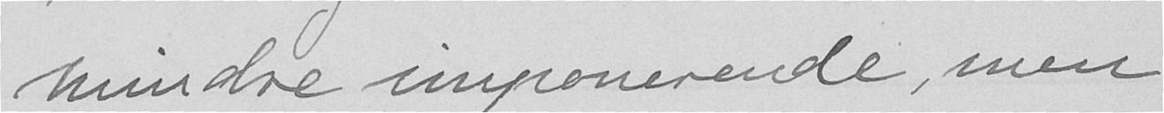mindre imponerende, men
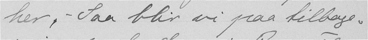her - Saa blir vi paa tilbage
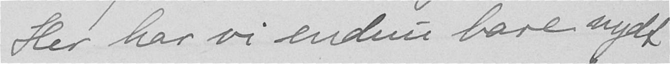Her har vi endnu bare nydt
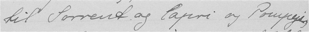til Sorrent og Capri og Pompeii
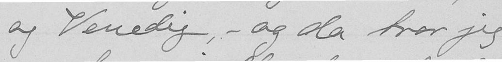og Venedig,- og da tror jeg
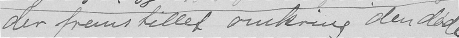der fremstillet omkring den dødes
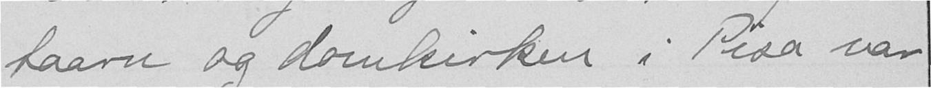taarn og domkirken i Pisa var
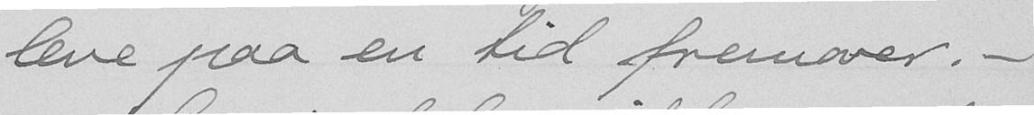leve paa en tid fremover. -
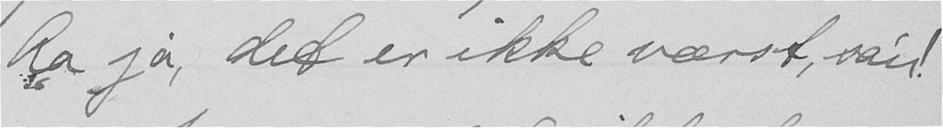Aa ja, det er ikke værst, sa'n!
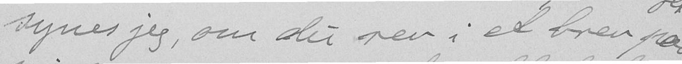synes jeg, om du rev i et brev paa
